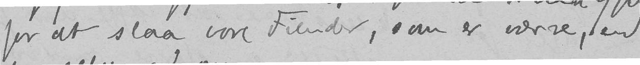for at slaa vore Fiender, som er værre, end
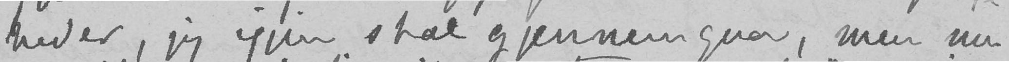heder, jeg igjen skal gjennemgaa, men nu
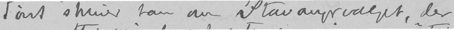Først skriver han om Stavangervalget, der
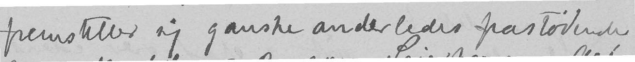fremstiller sig ganske anderledes frastødende
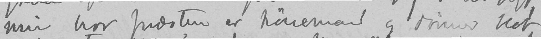Min bror præsten er høiremand og dømmer blot
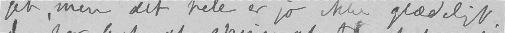get, men det hele er jo ikke glædeligt.
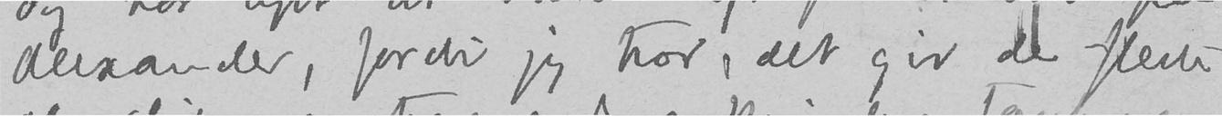Alexander, fordi jeg tror, det gir de fleste
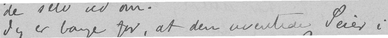Jeg er bange for, at den uventede Seier i
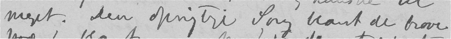meget. Den oprigtige Sorg blant de brave
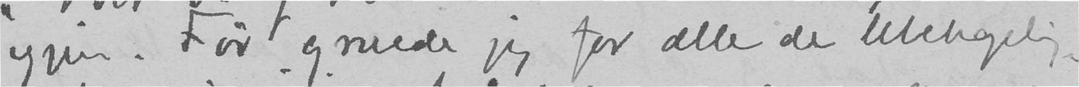igjen. Før gruede jeg for alle de ubehagelig-
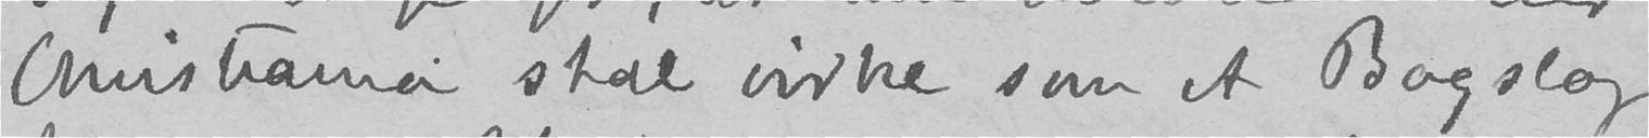Christiania skal virke som et Bagslag
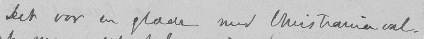Det var en glæde med Christiania val-
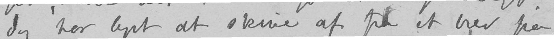Jeg har lyst at skrive af fra et brev fra
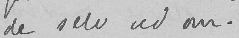de selv ved om.
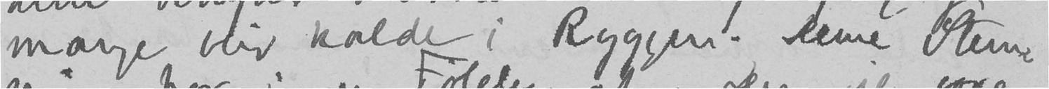mange blir kalde i Ryggen. Denne Stem-
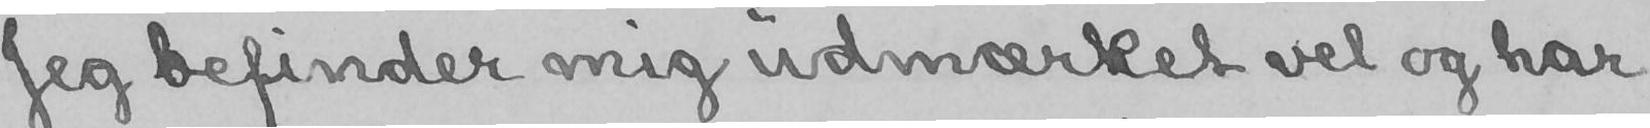Jeg befinder mig udmærket vel og har
